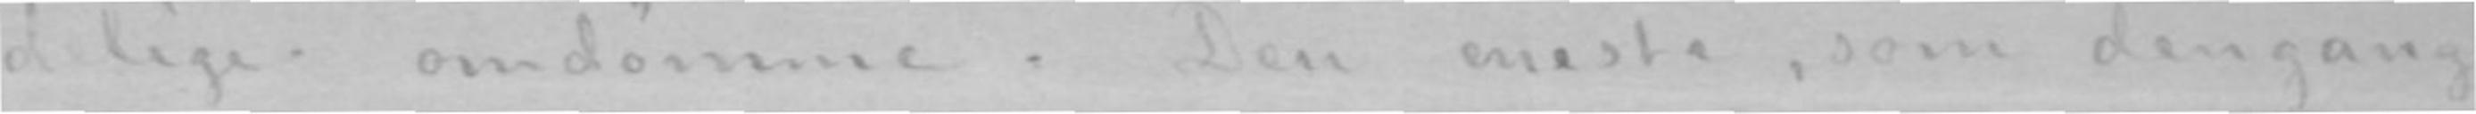delige omdømme. Den eneste, som dengang
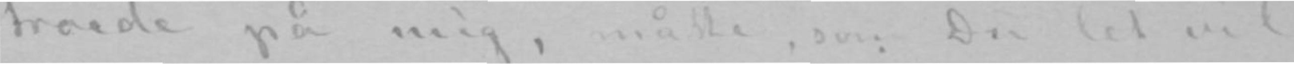troede på mig, måtte, som Du let vil
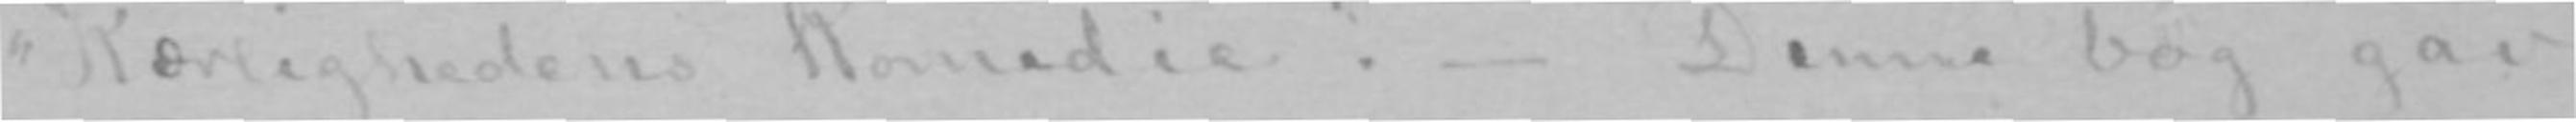«Kærlighedens Komedie».- Denne bog gav
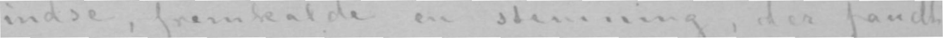indse, fremkalde en stemning, der fandt
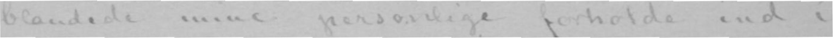blandede mine personlige forholde ind i
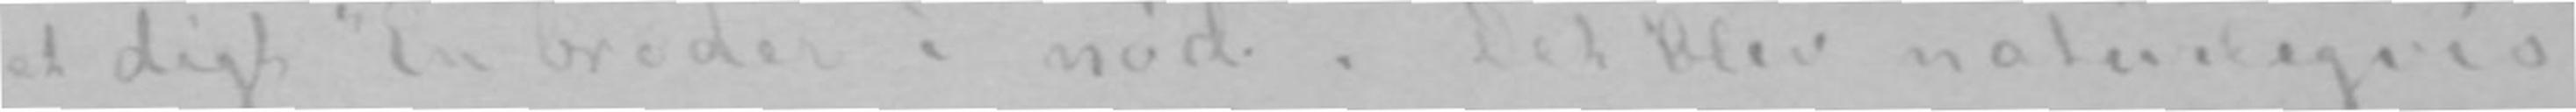et digt «En broder i nød. Det blev naturligvis
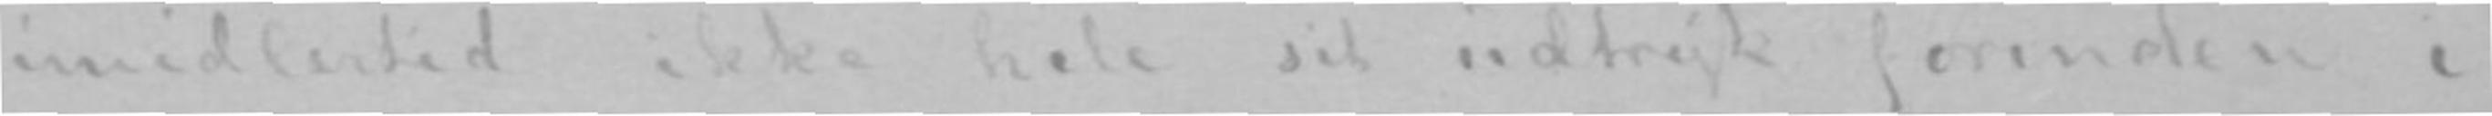imidlertid ikke hele sit udtryk forinden i
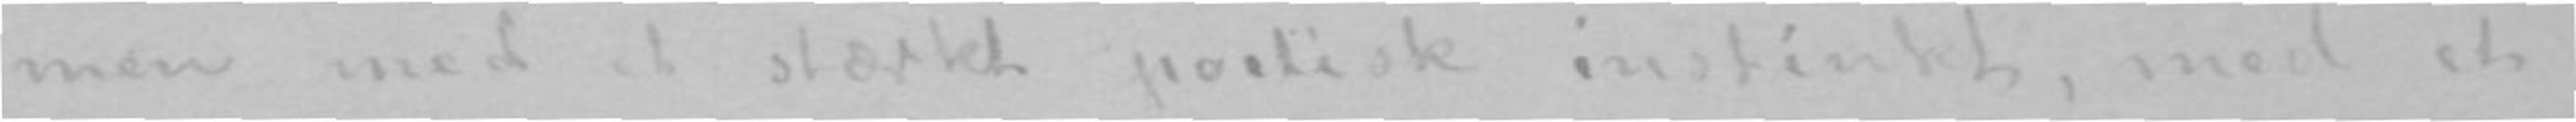men med et stærkt poetisk instinkt, med et
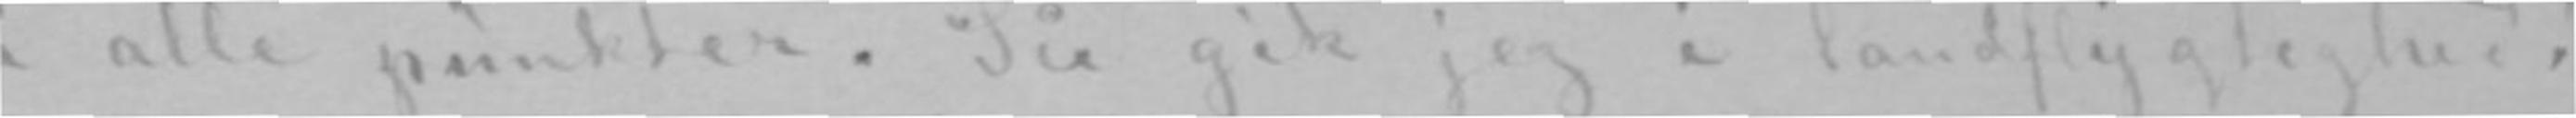på alle punkter. Så gik jeg i landflygtighed!
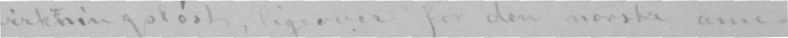virkningsløst, ligeover for den norske ame-
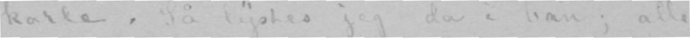karle. Så lystes jeg da i ban; alle var
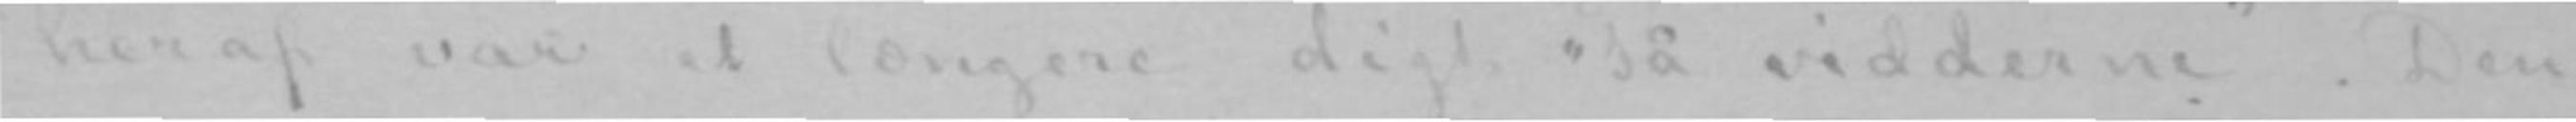heraf var et længere digt «På vidderne». Den
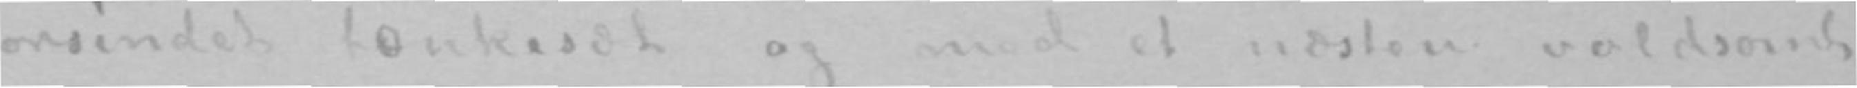storsindet tænkesæt og med et næsten voldsomt
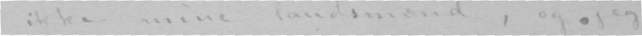forstod ikke mine landsmænd, og jeg
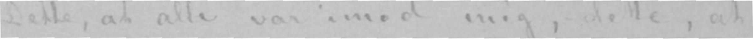Dette, at alle var imod mig,- dette, at
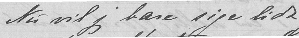Nu vil jeg bare sige lidt
